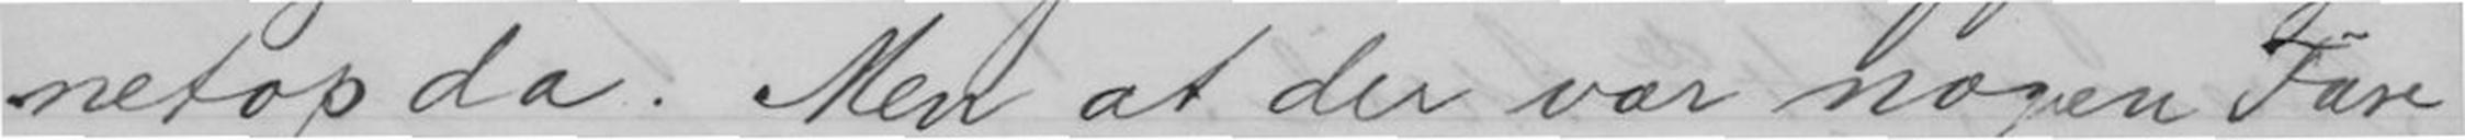netop da. Men at der var nogen Fare
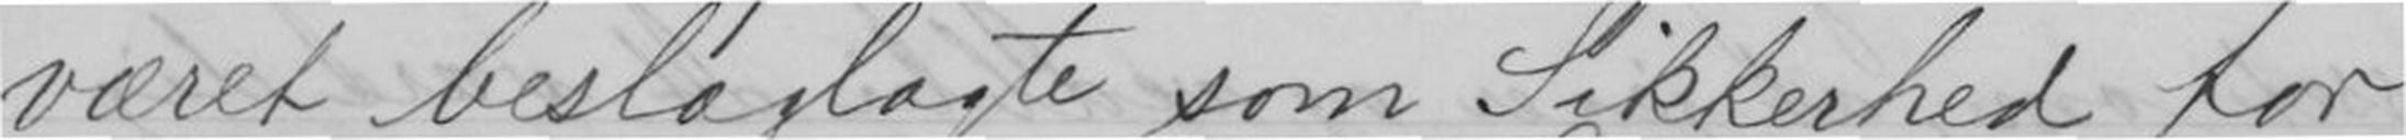været beslaglagte som Sikkerhed for
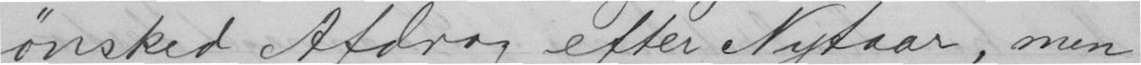ønsked Afdrag efter Nytaar, men
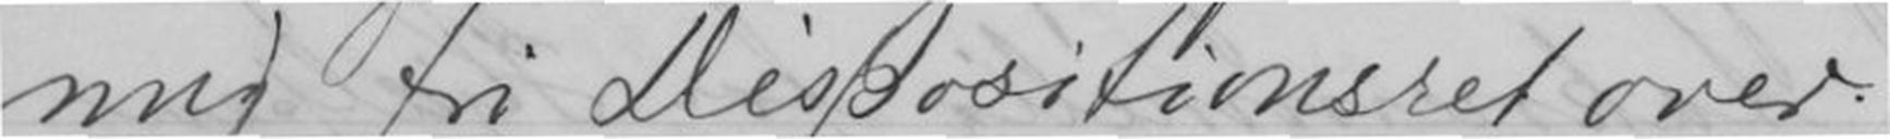mig fri Dispositionsret over.
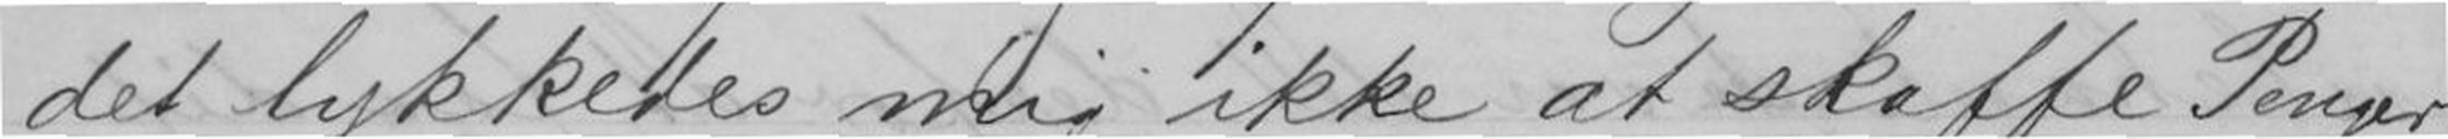det lykkedes mig ikke at skaffe Penger
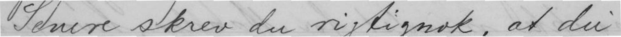Senere skrev du rigtignok, at du
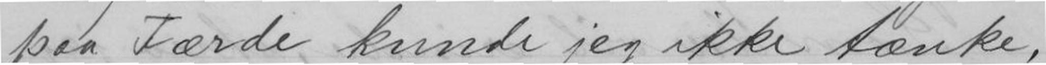paa Ferde kunde jeg ikke tænke,
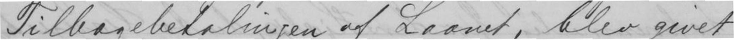Tilbagebetaligen af Laanet, blev givet
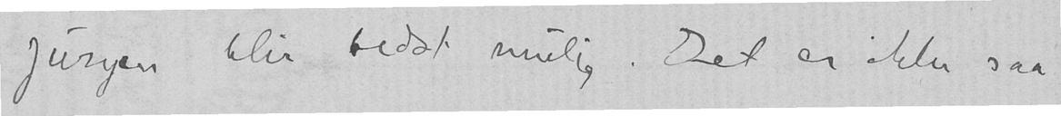Juryen blir bedst mulig. Det er ikke saa
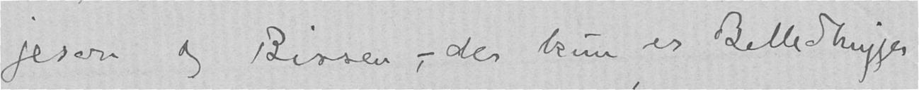jesen og Bissen - der kun er Billedhugger
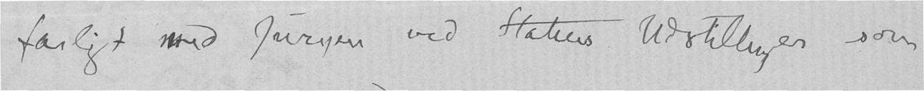farligt med Juryen ved Statens Udstilliger som
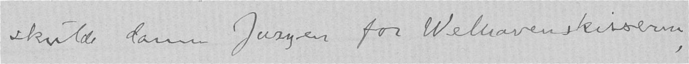skulde danne Juryen for Welhavenskissen,
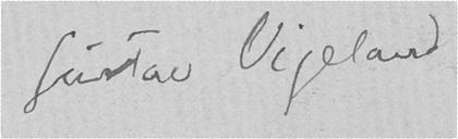Gustav Vigeland
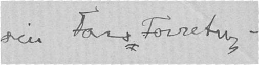sin Fars Forretning -
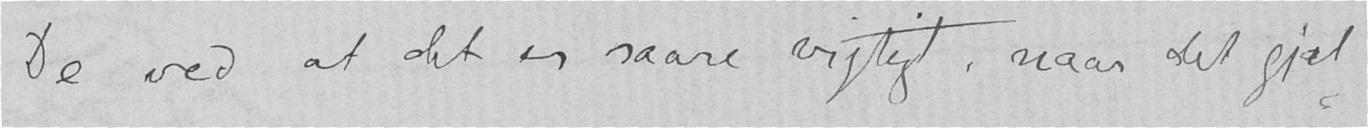De ved at det er saare vigtigt, naar det gjæl-
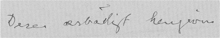Deres ærbødigt hengivne
